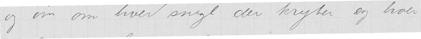og øm om hver snegl der kryber og hver
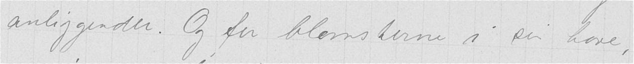anliggender. Og for blomsterne i sin have,
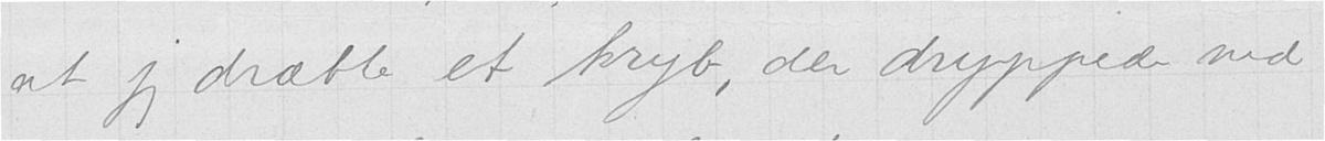at jeg dræbte et kryb, der dryppede ned
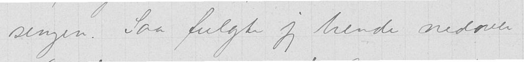sengen. Saa fulgte jeg hende nedover.
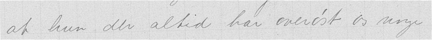at hun der altid har overøst os unge
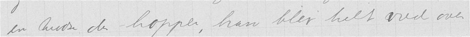en tudse der hopper, han blev helt vred over
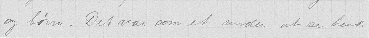og børn. Det var som et under at se hende
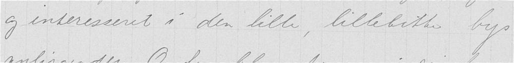og interesseret i den lille, lillebitte bys
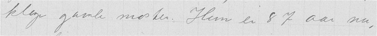kloke gamle moster. Hun er 87 aar nu,
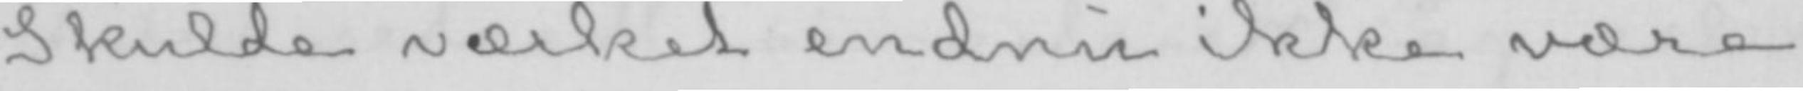Skulde værket endnu ikke være
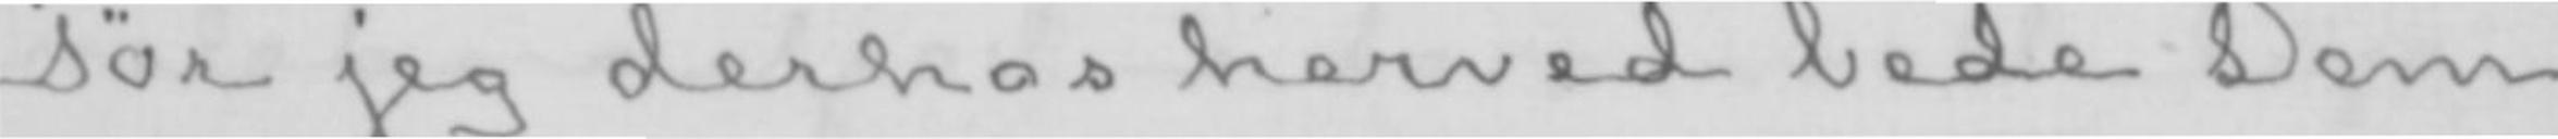Tør jeg derhos herved bede Dem
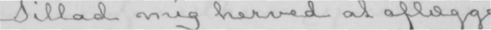Tillad mig herved at aflægge
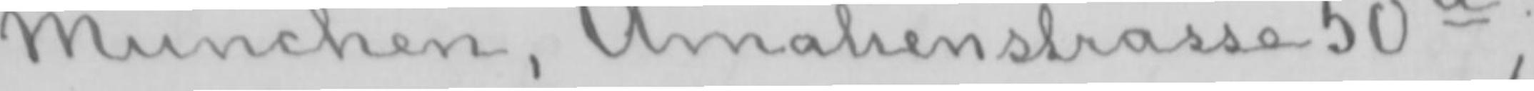München, Amalienstrasse 50a,
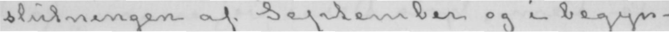slutningen af September og i begyn-
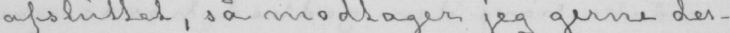afsluttet, så modtager jeg gerne der-
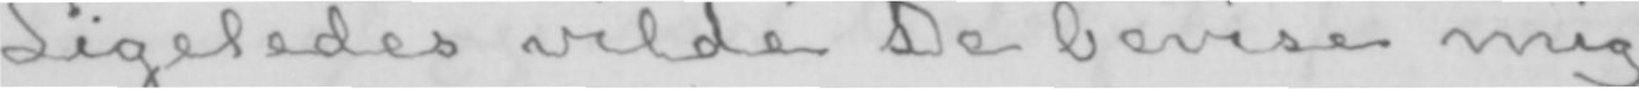Ligeledes vilde De bevise mig
